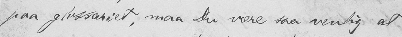paa glossariet, maa Du være saa venlig at
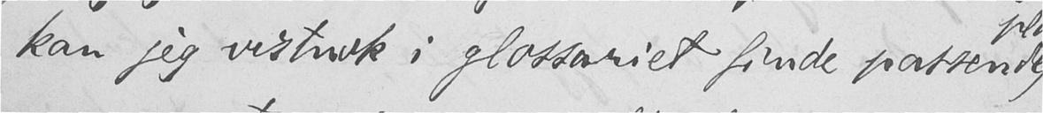kan jeg vistnok i glossariet finde passende
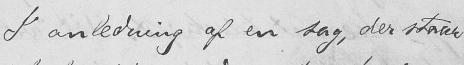I anledning af en sag, der staar
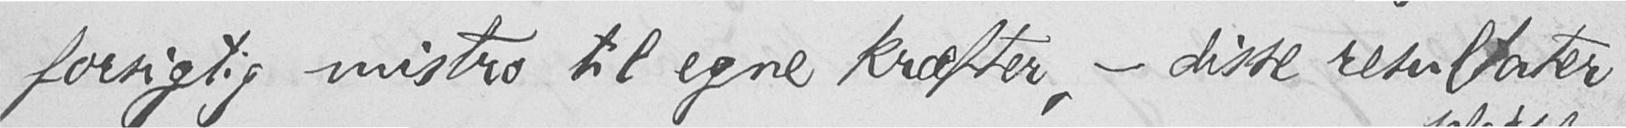forsigtig mistro til egne kræfter, - disse resultater
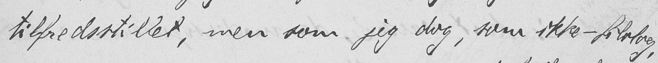tilfredsstillet, men som jeg dog, som ikke-filolog,
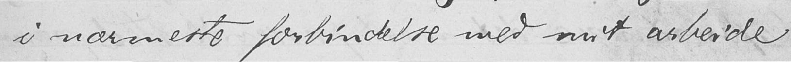i nærmeste forbindelse med mit arbeide
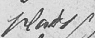plads
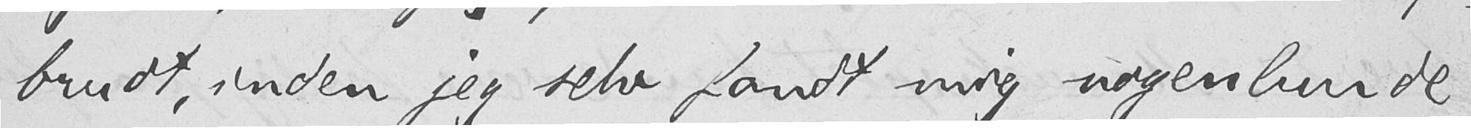brudt, inden jeg selv fandt mig nogenlunde
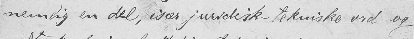nemlig en del, især juridisk-tekniske ord og
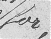for;
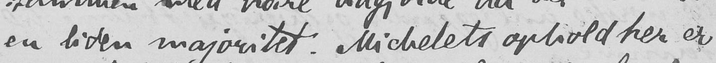en liden majoritet. Michelets ophold her er
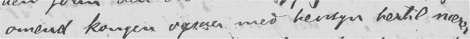omend kongen ogsaa med hensyn hertil nærer
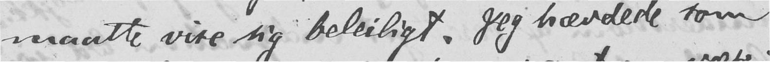maatte vise sig beleiligt. Jeg hævdede som
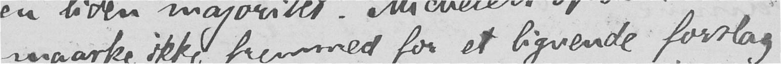maaske ikke fremmed for et lignende forslag
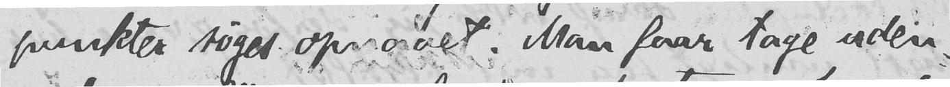punkter søges opnaaet. Man faar tage uden-
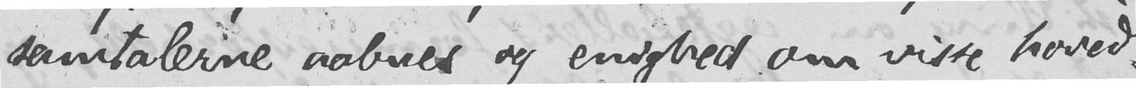samtalerne aabnes og enighed om visse hoved-
